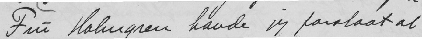Fru Holmgren havde jeg forstaat at
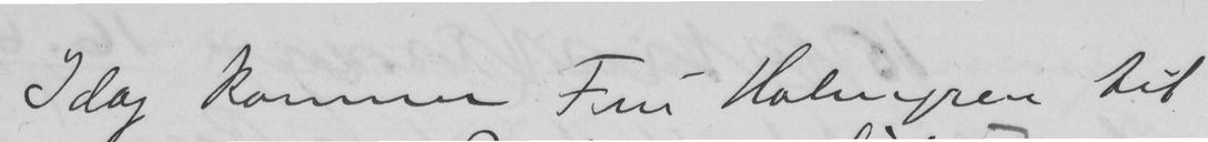Idag komme Fnu Holmgen til
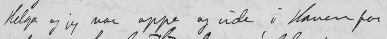Helga og jeg var oppe og ude i Haven for
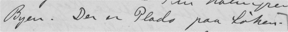Byen. Der er Plads paa Løken.
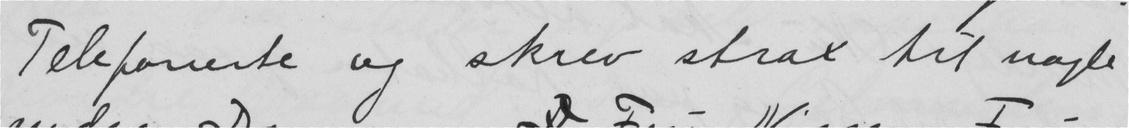Telefonerte og skrev strax til nogle
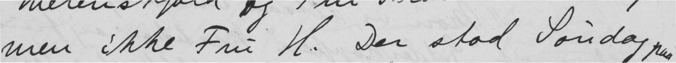men ikke Fru H. Der stod Søndag paa
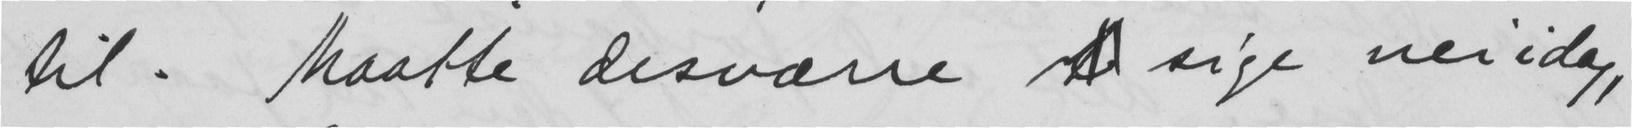til. Maatte desværre  sige nei idag,
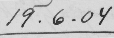19.6.04
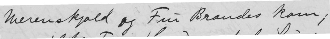Werenskjold og Fru Brandes kom;
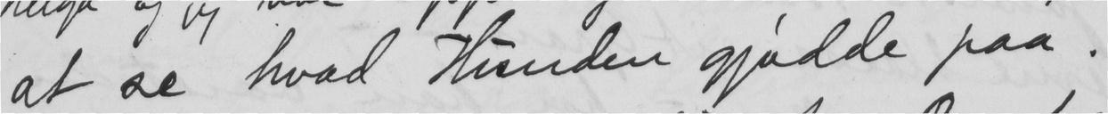at se hvad Hunden gjødde paa.
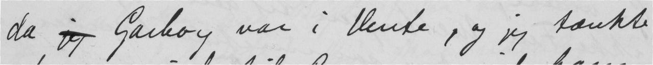da  Garborg var i Vente, og jeg tænkte
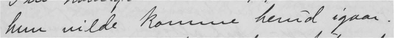hun vilde komme herud igaar.
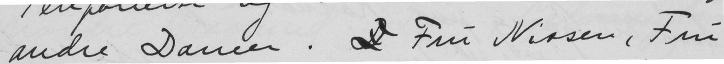andre Damer.  Fru Nissen, Fru
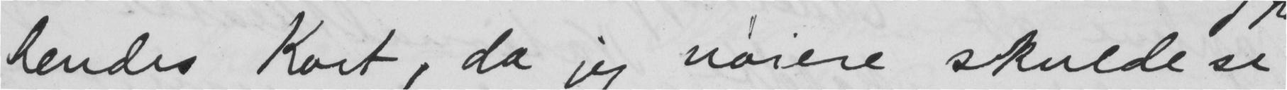hendes Kort, da jeg nøiere skulde se
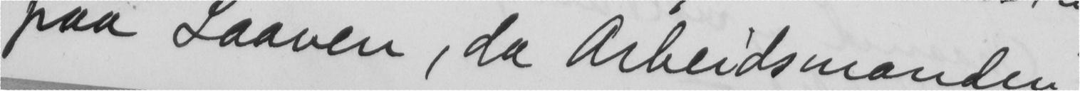paa Laaven, da Arbeidsmanden
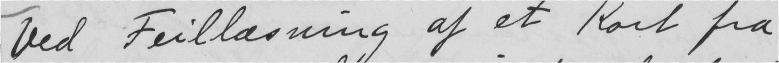Ved Feillæsning af et Kort fra
